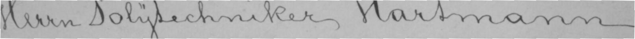Herrn Polytechniker Hartmann
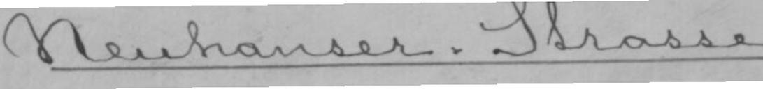Neuhauser-Strasse.
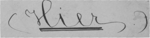(Hier.)
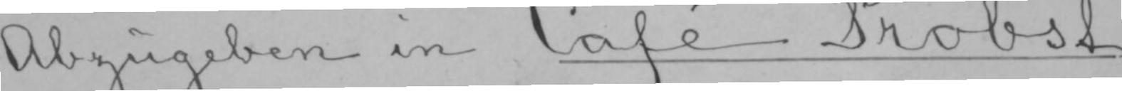Abzugeben in Café Probst.
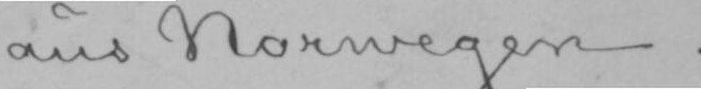aus Norwegen.
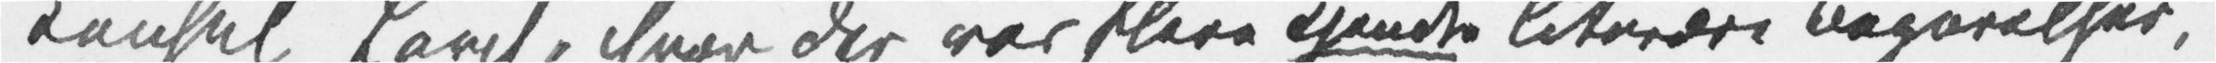Konsul Lorch, hvor der var flere kjendte literære Begavelser,
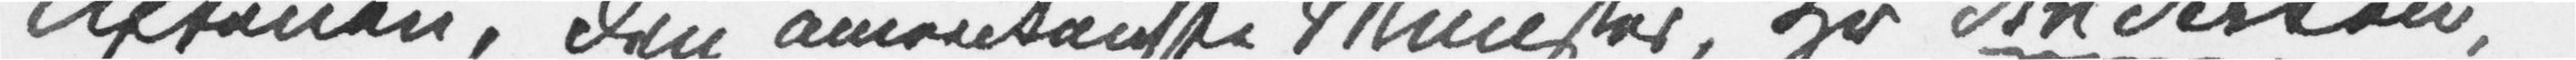Aftenen, den amerikanske Minister, Hr Anderson,
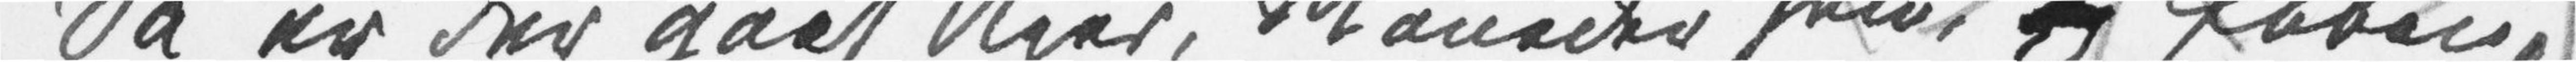Så er der gået Uger, Måneder hen, og Ebben,
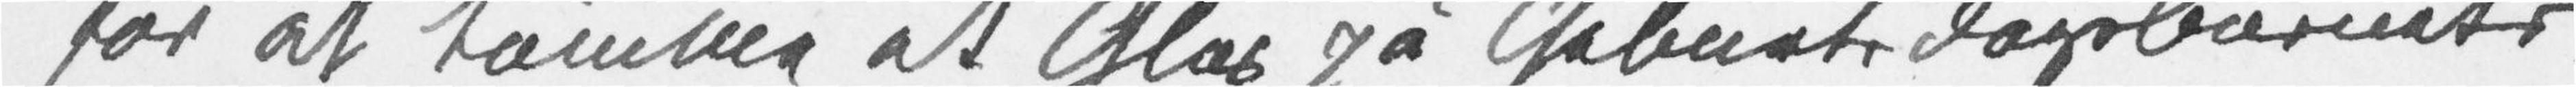for at tømme et Glas på Geburtsdagsbarnets
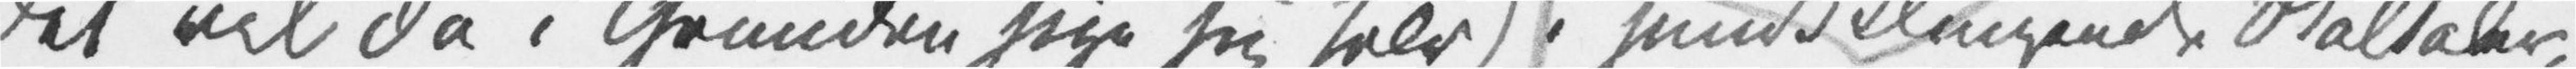(det vil da i Grunden sige sig selv) i smukklingende Skåltaler.
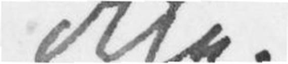ikke.
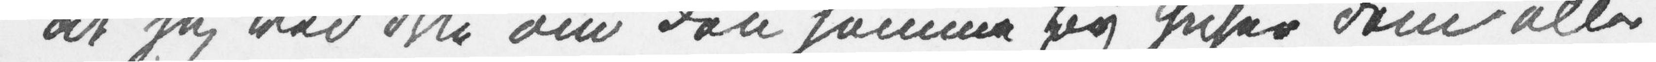at jeg ved ikke om den samme By huser dem eller
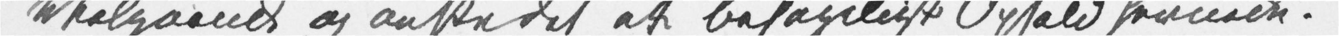Velgående og ønske det «et behageligt Ophold hernede».
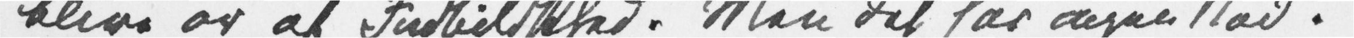blive ør af Indbildskhed. Men det har ingen Nød.
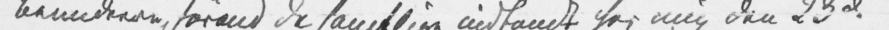Beundrere, førend de samtlige indfandt [sig] hos mig den 23de
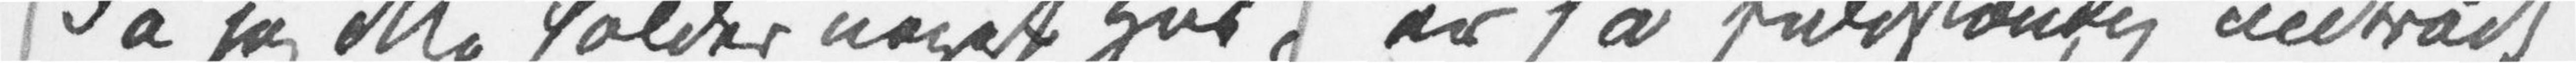da jeg ikke holder noget Hus, er så fuldstændig indtrådt
